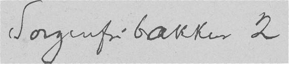Sorgenfribakken 2
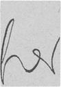hv
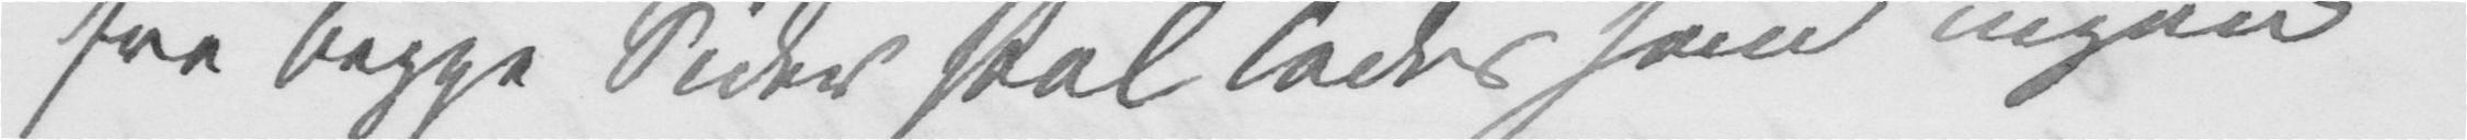fra begge Sider skal lades som ingen
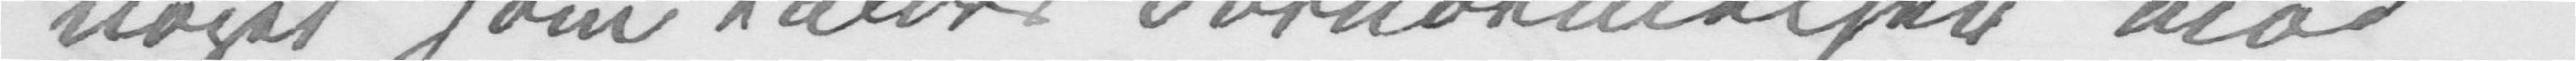noget som kaldes Fornærmelser mod
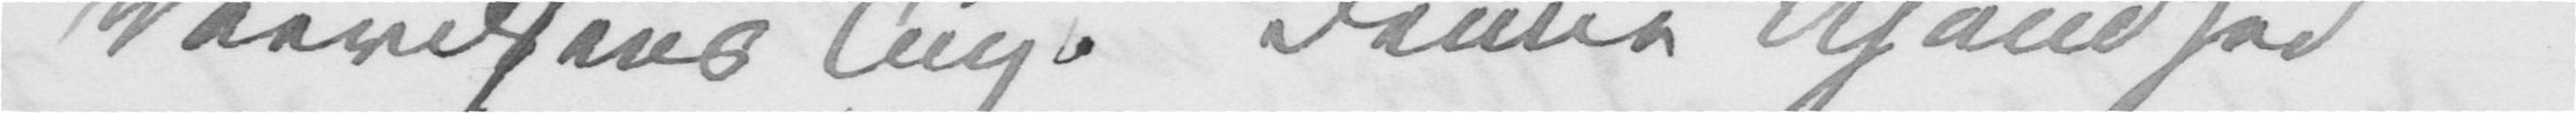Verdsens Ting. Denne Usandhed
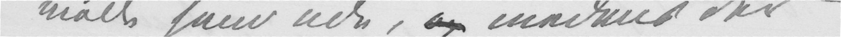møde ham ude, og medens der
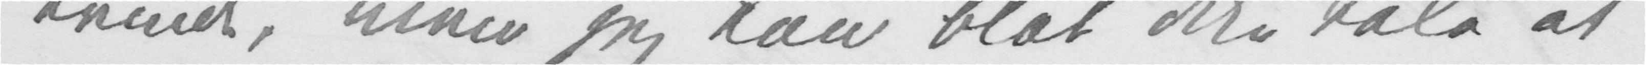Kvinde, men jeg kan blot ikke tåle at
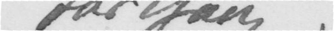for Gang
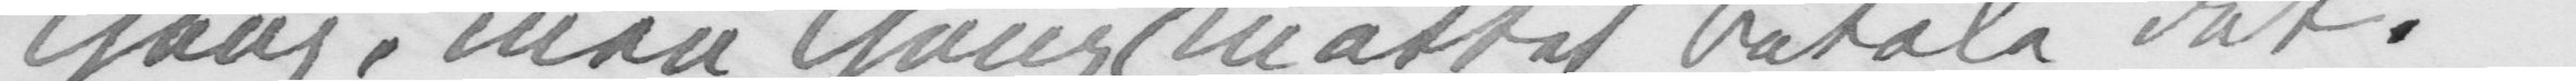Gang, men Gang måttet betale det.
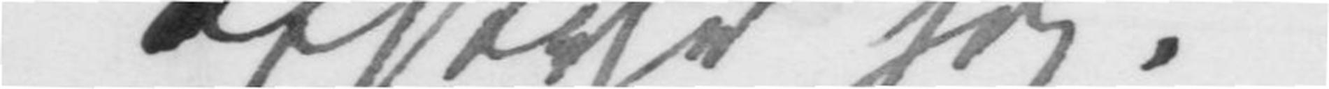afskyr jeg.
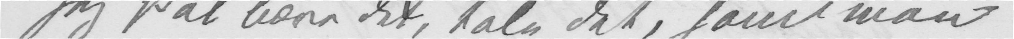Jeg skal bære det, tåle det, som man
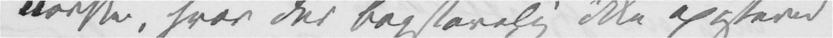Norske, hvor der bogstavelig ikke existerer
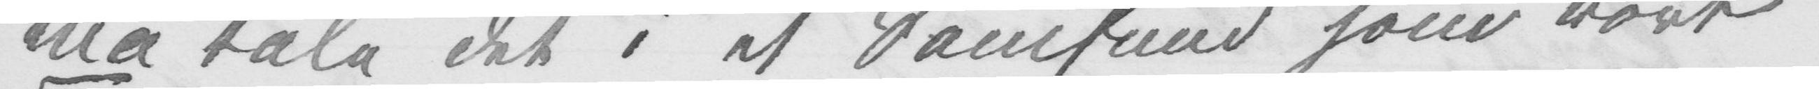må tåle det i et Samfund som vort
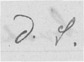D.S
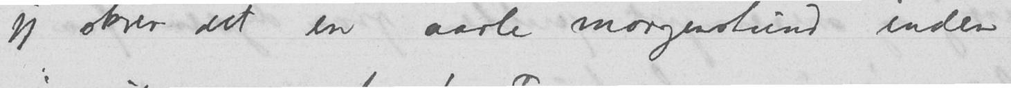jeg skrev det en aarle morgenstund inden
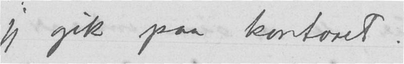jeg gik paa kontoret.
